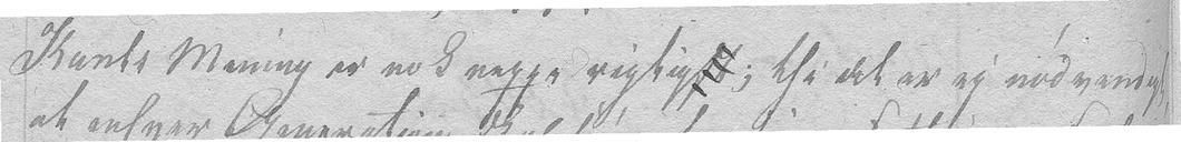Kants Mening er nok neppe rigtig; thi det er ey nødvendigt
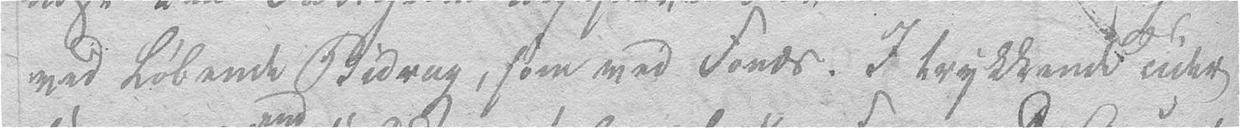ved løbende Bidrag, som ved Fonds. I trykkende Tider
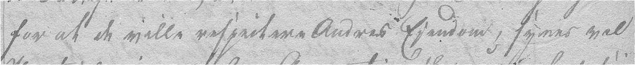for at de ville respectere andres Eiendom; synes vel
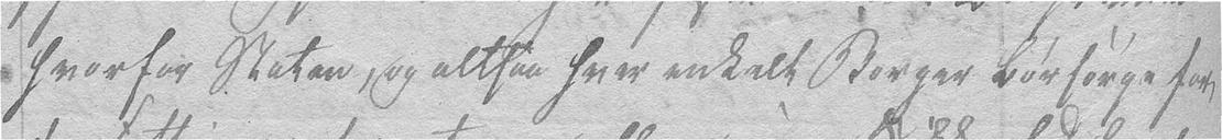hvorfor Staten, og altsaa hver enkelt Borger bør sørge for
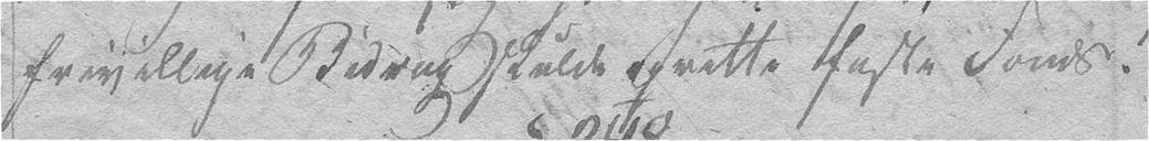frivillige Bidrag skulde oprette faste Fonds.
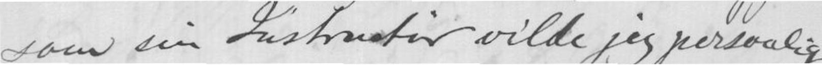som sin Instrutør vilde jeg personlig
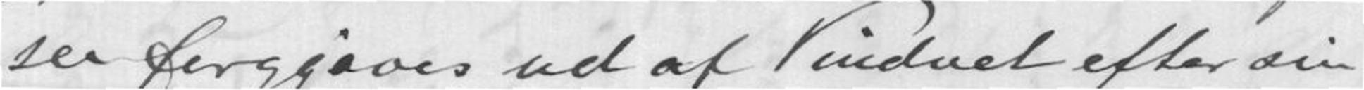see forgeves ud af Vinduet efter sin
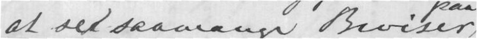at see saamange Beviser
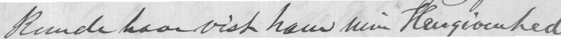kunde have vist ham min Hengivenhed -
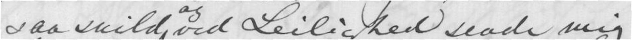saa snild ved Leilighed sende mig
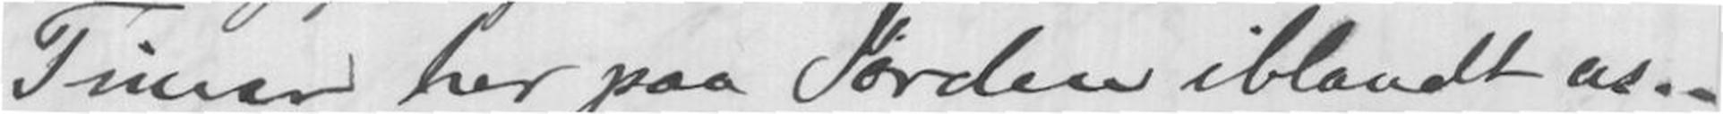Timer her paa Jorden iblandt os. -
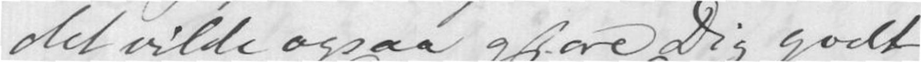det vilde ogsaa gjøre Dig godt
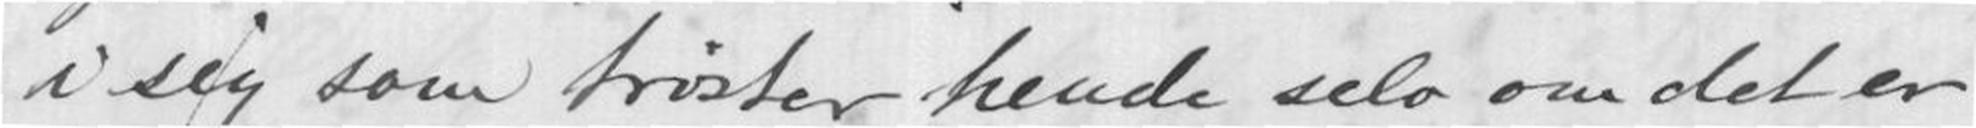i sig som trøster hende selv om det er
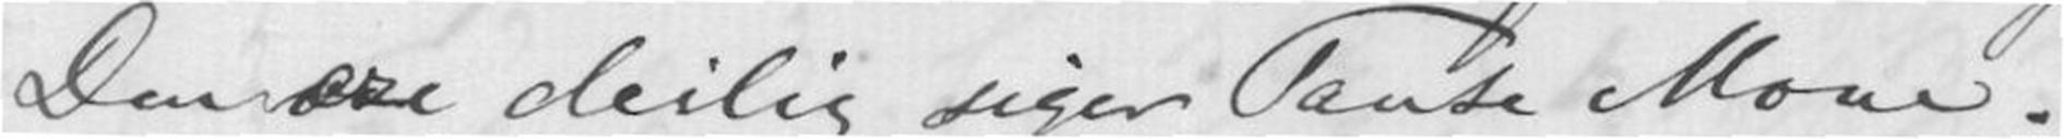Den ere deilig siger Tante Mone.
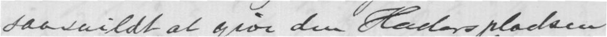saa snildt at give den Hæderspladsen
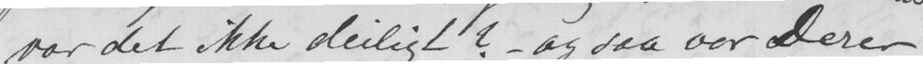var det ikke deiligt? - og saa var Derer
